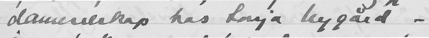dameselskap has Sonja Nygård -
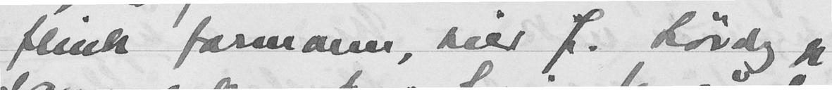flink formann, sier J. Lørdag jeg i
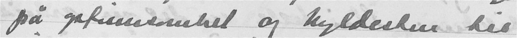på opfinnsomhet og hyldesten til
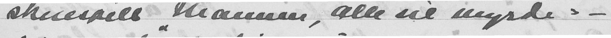skuespill "Mannen, alle vil myrde" -
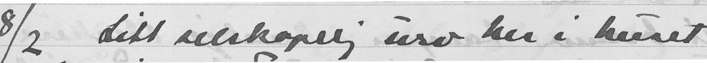8/2 Litt selskapelig uro her i huset
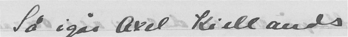Så igår Axel Kiellands
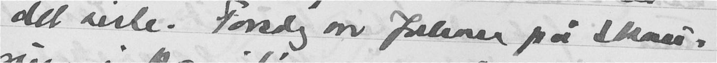det siste. Torsdag var Johan på Skau-
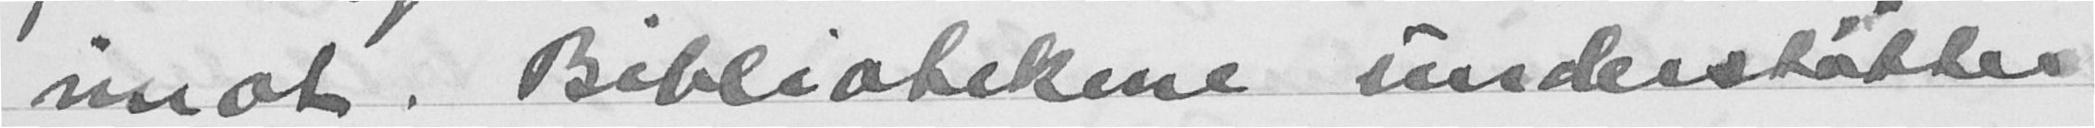imot. Bibliotekene understøtter
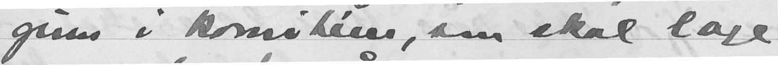gum i komitéen, som skal lage
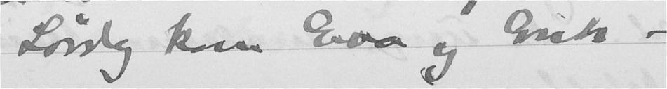Lørdag kom Eva og Erik -
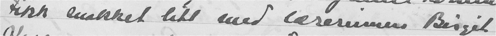Fikk snakket litt med lærerinnen Birgit
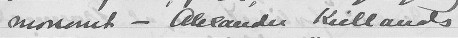morsomt - Alexander Kiellands
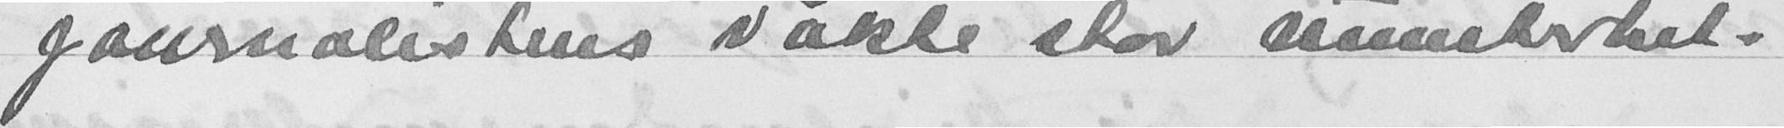journalistene vakte stor munterhet.
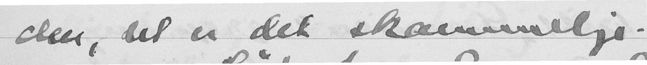den, det er det skammelige.
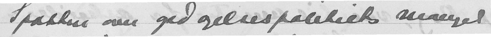Spalten over opdagelsespolitiets mangel
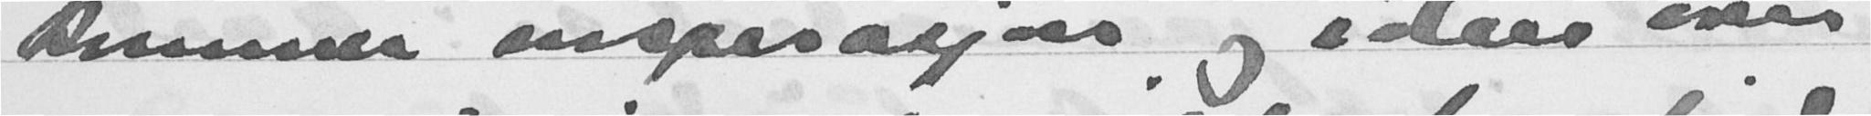kommer inspirasjon og idéen over
